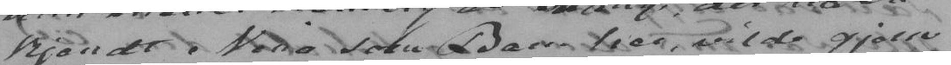kjændt Nina som Barn her, vilde gjerne
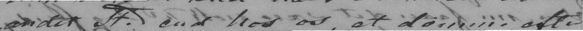andet Sted end hos os, at dømme efter
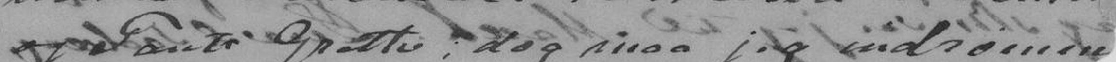og Tante Grethe; dog maa jeg indrømme
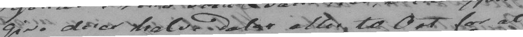give deres halve Daler eller to Ort for at
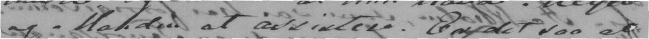og Manden at assistere. Er det saa at
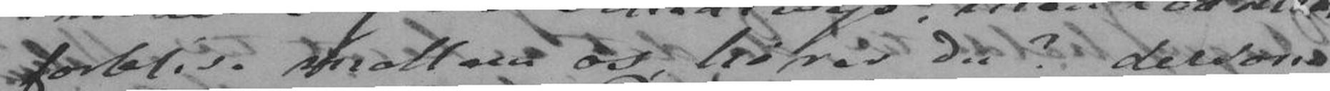forblive mellem os, hører Du? dersom
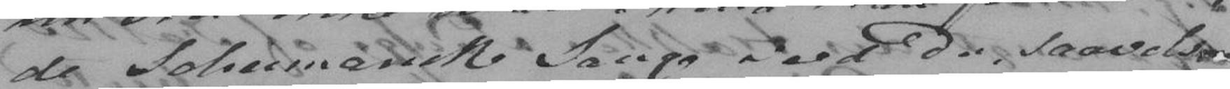de Schumanske Sange veed Du, saavelsom
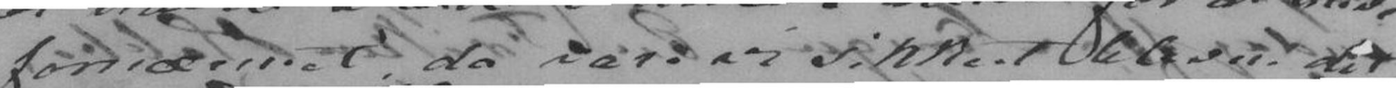fornærmet, da være vi sikkert blevne det
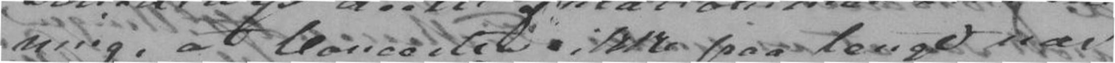ning, at Concerten ikke paa langt nær
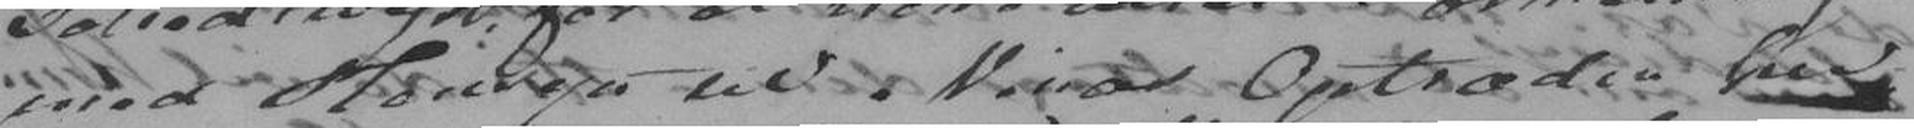med Hensyn til Ninas Optræden her
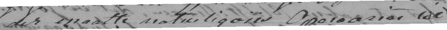der maatte naturligviis Operaarier til
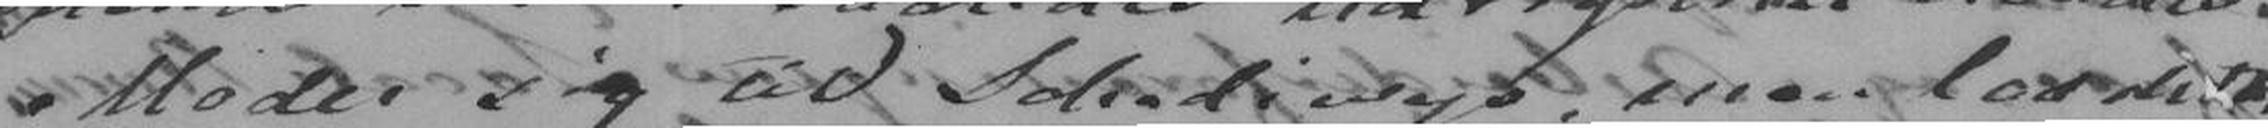Moder sig til Schediwys, men lad dette
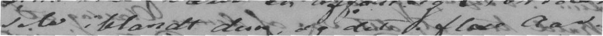selv blandt dem, og det i flere Aar.
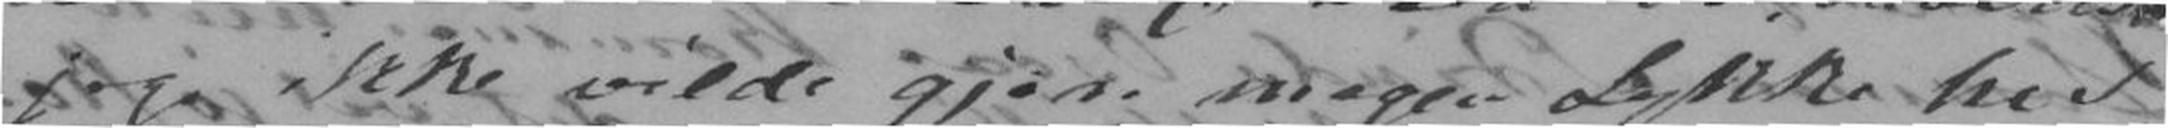jeg ikke vilde gjøre megen Lykke her,
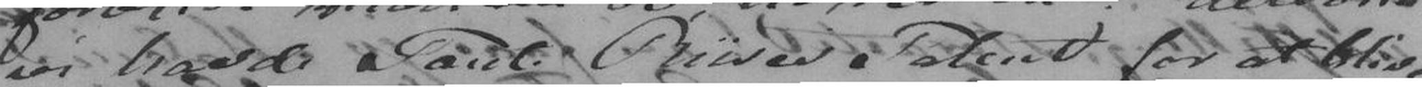vi havde Tante Riises Talent for at blive
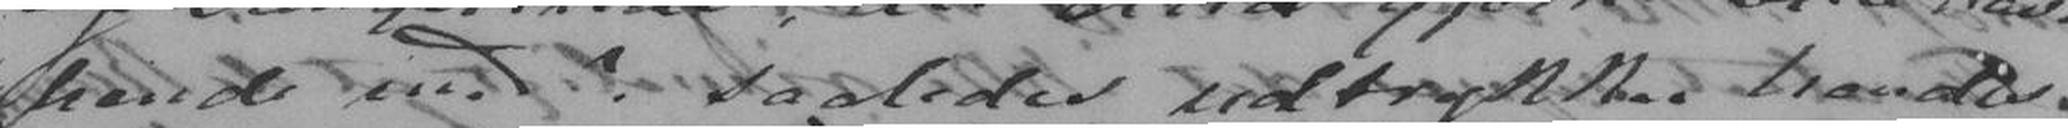hende med? saaledes udtrykker hendes
